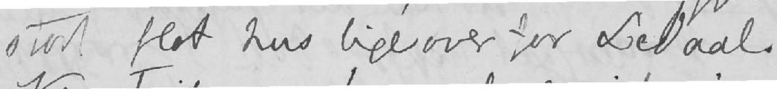stort flot hus ligeoverfor Ledaal.
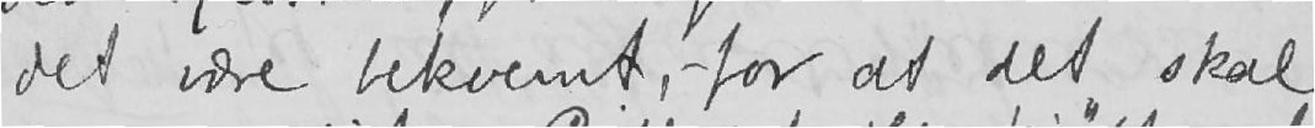det være bekvemt, for at det skal
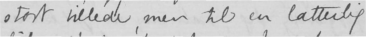stort billede, men til en latterlig
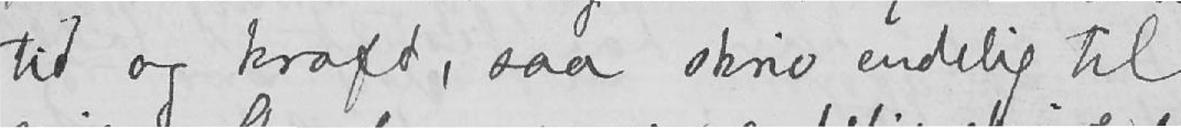tid og kraft, saa skriv endelig til
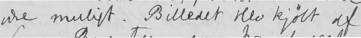være muligt. Billedet blev kjøbt af
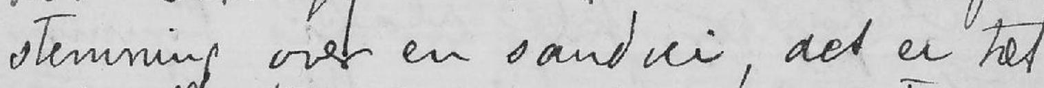stemning over en sandvei, det er tæt
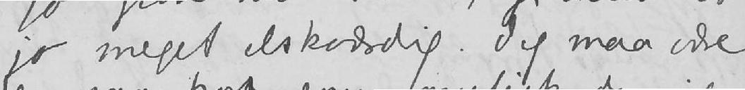jo meget elskværdig. Jeg maa være
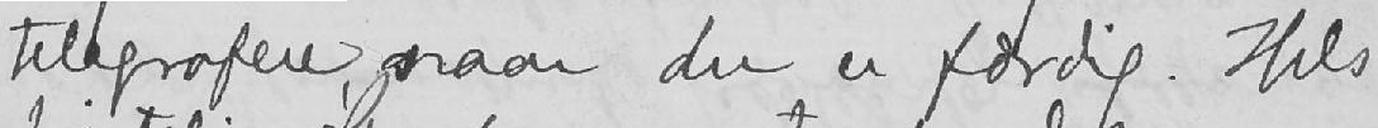telegrafere naar du er færdig. Hils
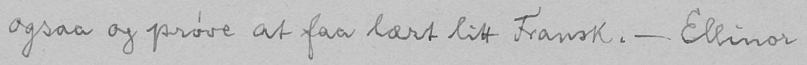ogsaa og prøve at faa lært litt Fransk. - Ellinor
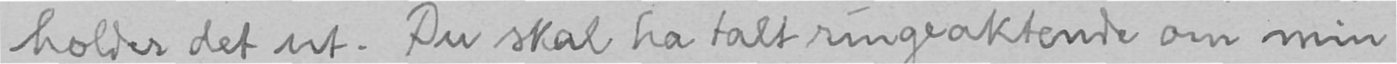holder det ut. Du skal ha talt ringeaktende om min
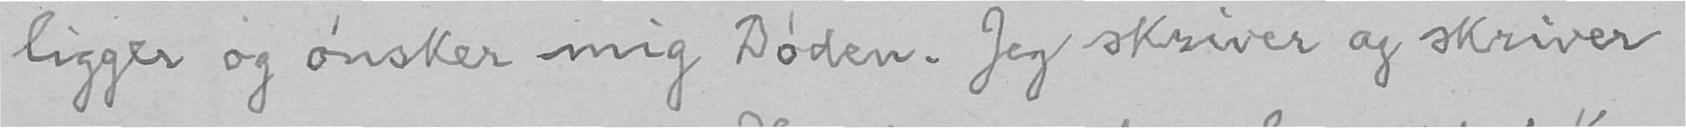ligger og ønsker mig Døden. Jeg skriver og skriver
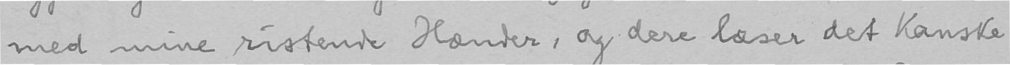med mine ristende Hænder, og dere læser det kanske
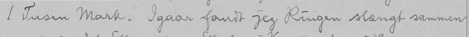1 Tusen Mark. Igaar fandt jeg Ringen slængt sammen
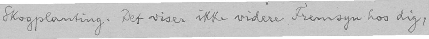Skogplanting. Det viser ikke videre Fremsyn hos dig,
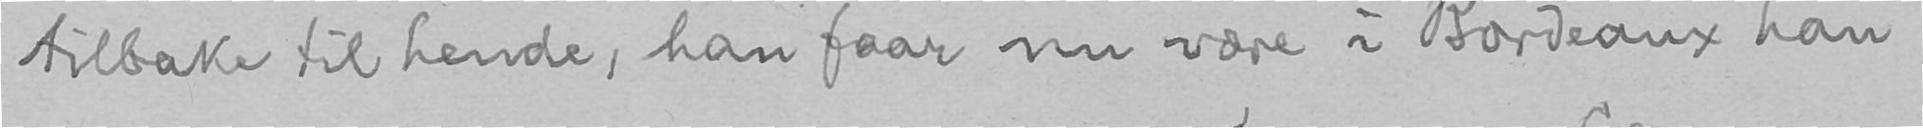tilbake til hende, han faar nu være i Bordeaux han
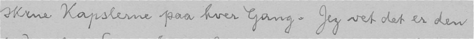skrue Kapslerne paa hver Gang. Jeg vet det er den
